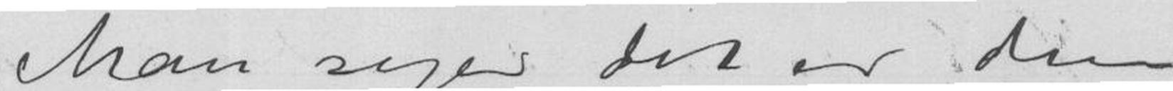Man siger det er den
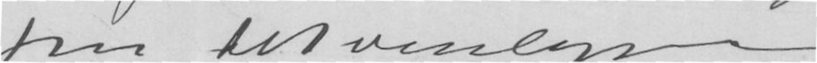paa det venligste
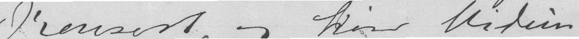Konsert og høre Vidun-
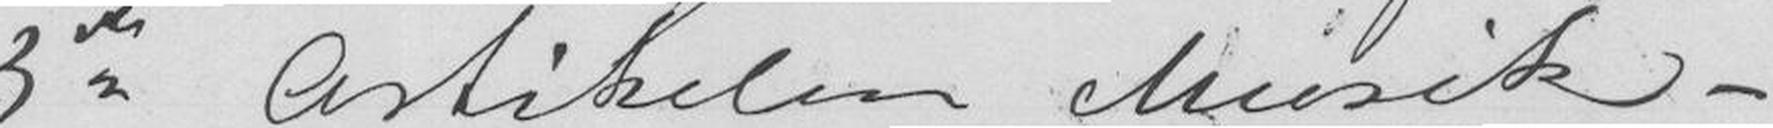3die Artikelen Musik -
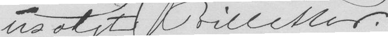usolgte Billetter.
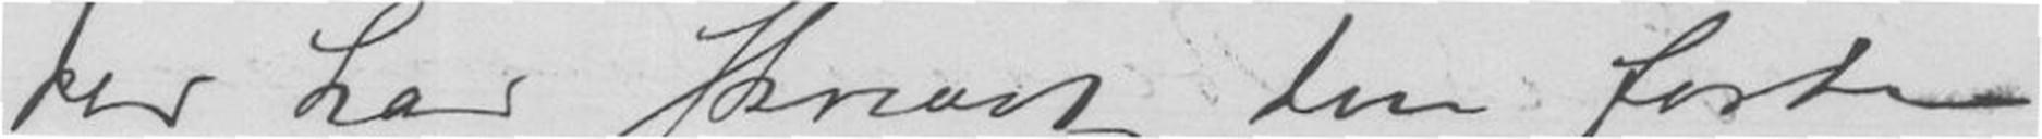der har skrevet den første
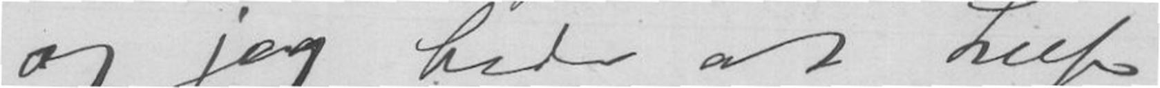og jeg beder at hilse
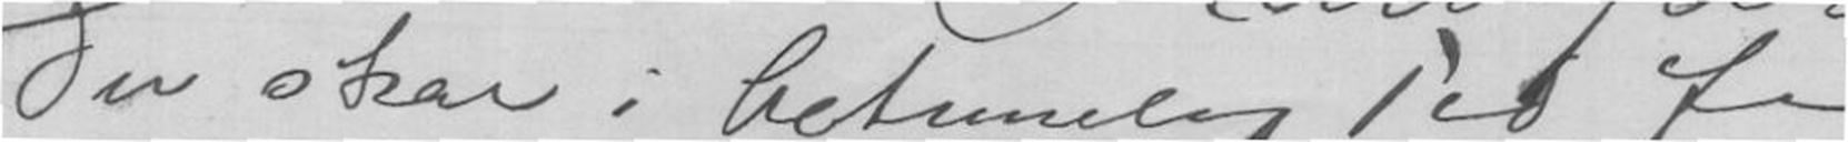Du skal i betimelig Tid faa
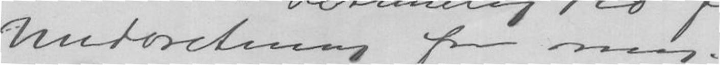Underetning fra mig.
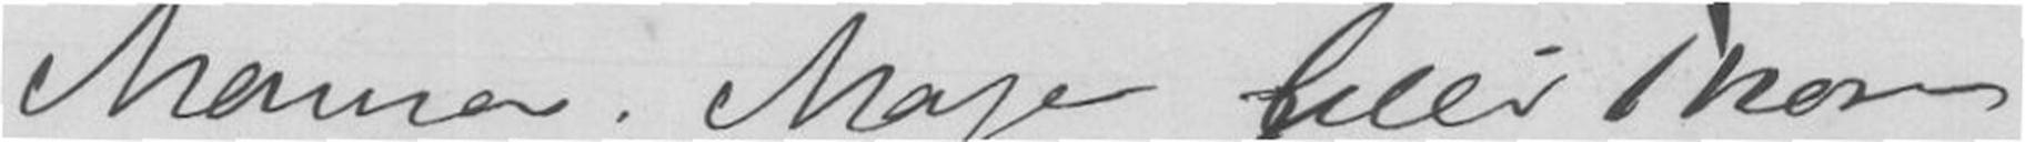Mama. Maje lille Thora
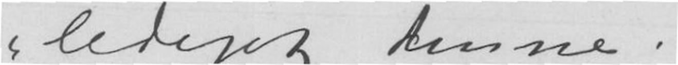lediget denne.
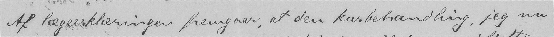Af lægeerklæringen fremgaar, at den kurbehandling, jeg nu
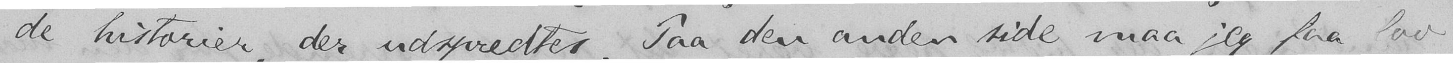de historier, der udspredtes. Paa den anden side maa jeg faa lov
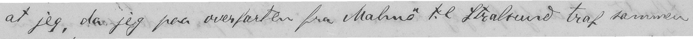at jeg, da jeg paa overfarten fra Malmö til Stralsund traf sammen
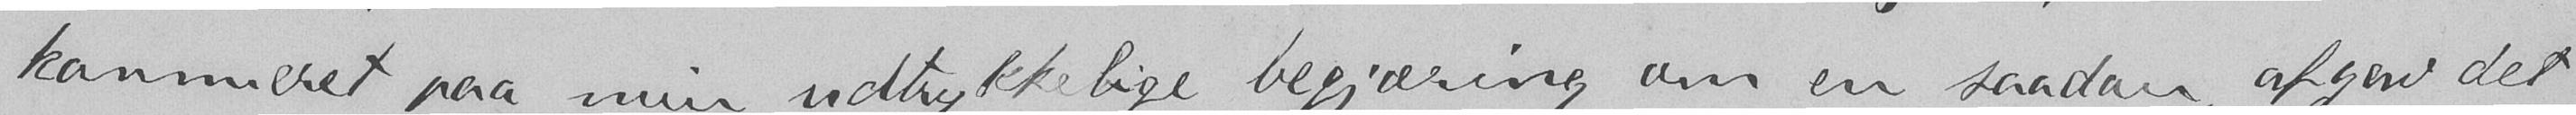kammeret paa min udtrykkelige begjæring om en saadan, afgav det
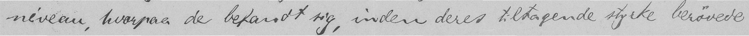niveau, hvorpaa de befandt sig, inden deres tiltagende styrke berøvede
